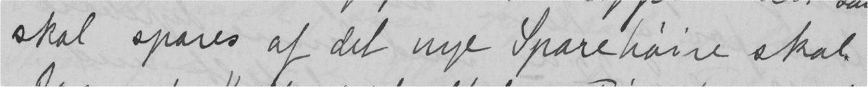skal spares at det nye Sparehøire skal
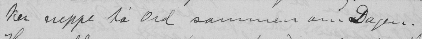ker neppe to Ord sammen om Dagen.
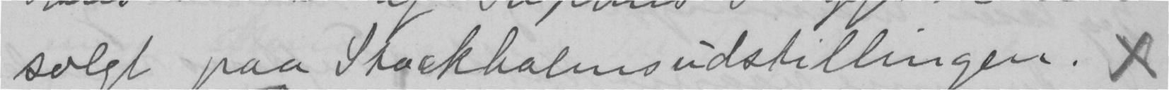solgt paa Stockholmsudstillingen. x
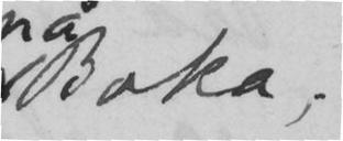Boka,
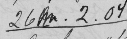26 .2.04
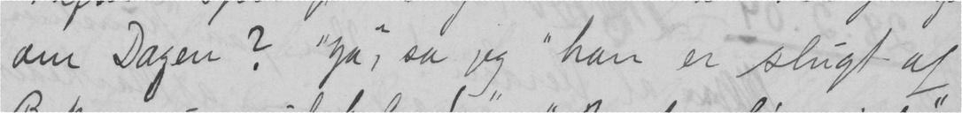om Dagen? "Ja", sa jeg "han er slugt af
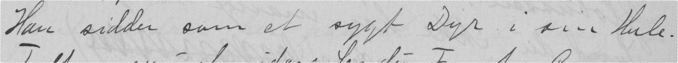Han sidder som et sygt Dyr i sin Hule.
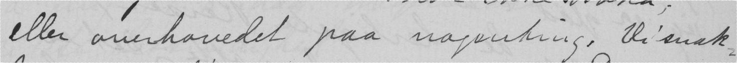eller overhovedet paa nogenting. Vi snak-
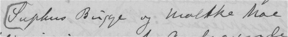Suphus Bugge og Moltke Moe
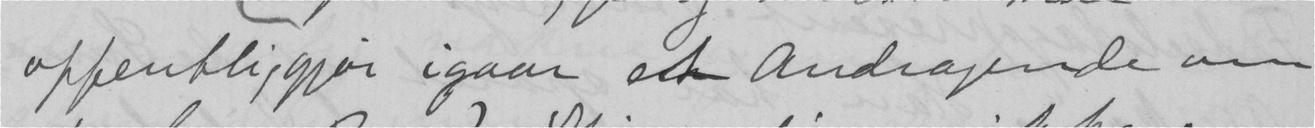offentliggjør igaar et Andragende om
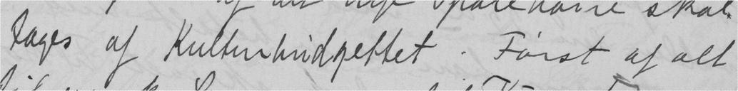tages af kulturbudgettet. Først af alt
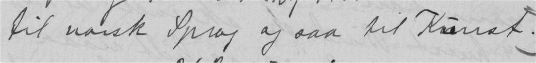til norsk Sprog og saa til Kunst.
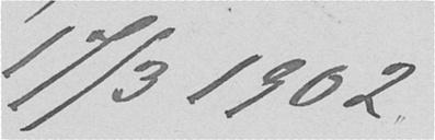17/3 1902
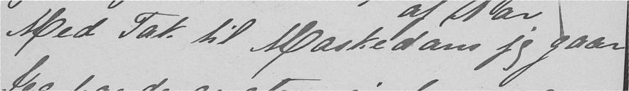Med Tak til Maskedans jeg gaar
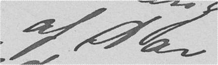af Aar
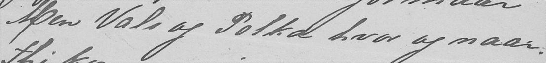Men Vals og Polka hvor og naar.
